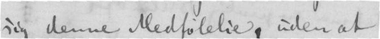sig denne Medfølelse, uden at
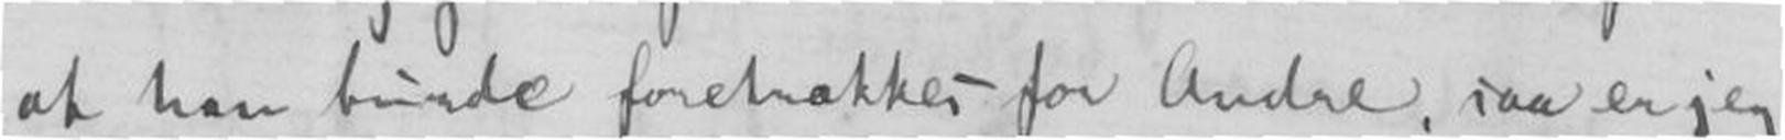at han burde foretrækkes for Andre, saa er jeg
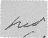hed
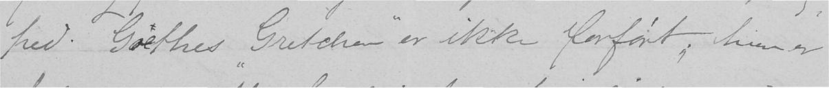hed. Goethes "Gretchen" er ikke forført; hun er
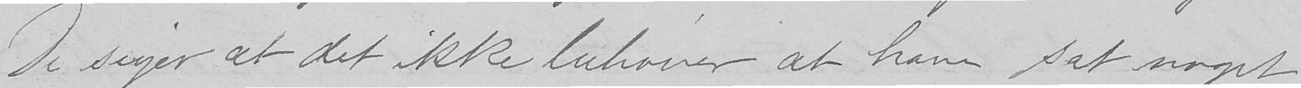De siger at det ikke behøver at have sat noget
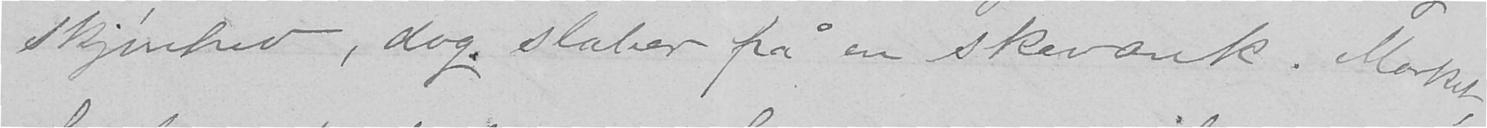skjønhed, dog slæber på en skavank. Mærket,
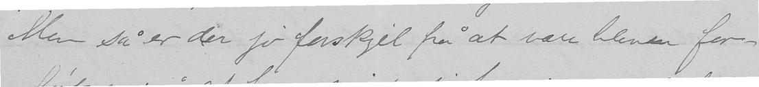Men så er der jo forskjel på at være bleven for-
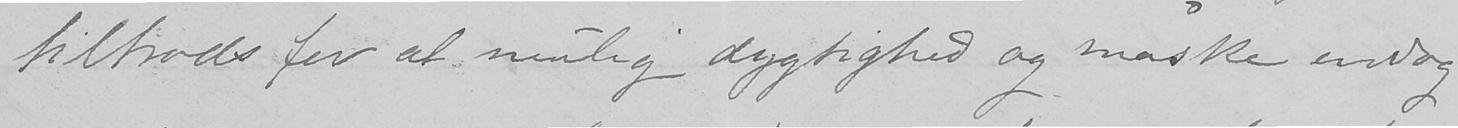tiltrods for al mulig dygtighed og måske endog
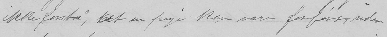ikke forstå, at en pige kan være forført, uden
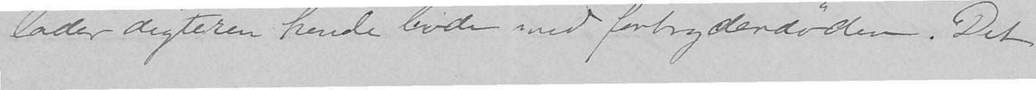lader digteren hende bøde med forbrydendøden. Det
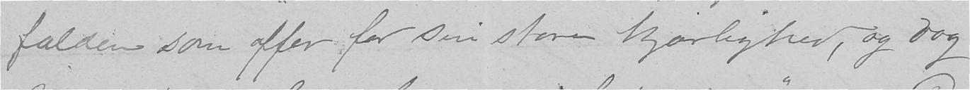falden som offer for sin store kjærlighed, og dog
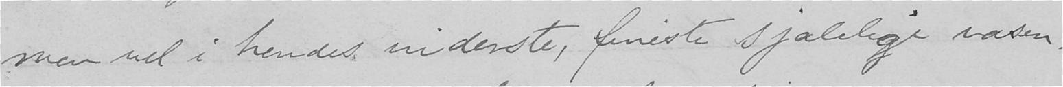men vel i hendes inderste, fineste sjælelige væsen.
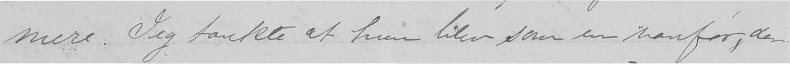mere. Jeg tænkte at hun blev som en vanfør, der
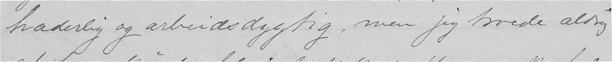hæderlig og arbeidsdygtig, men jeg troede aldrig
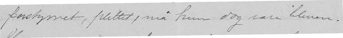forstyrret, plettet, må hun dog være bleven.
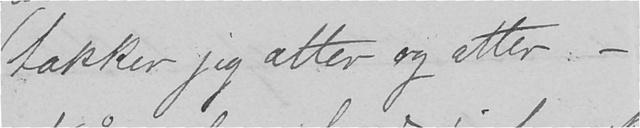takker jeg atter og atter. -
